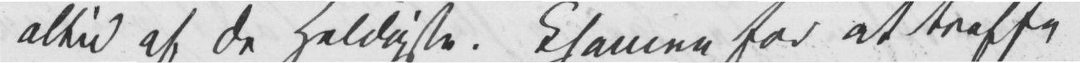altid af de Heldigste. Chancen for at treffe
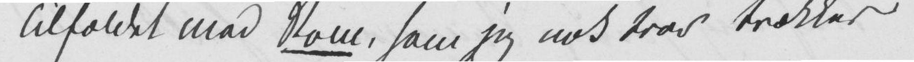Tilfældet med Rom, som jeg nok tror trækker
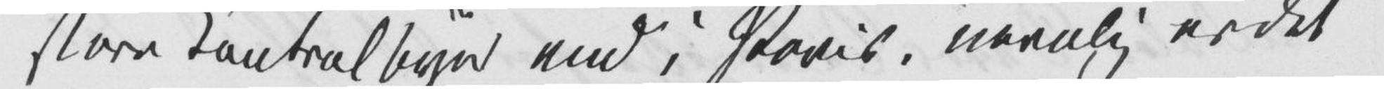store Centralbyer end i Paris, navnlig er det
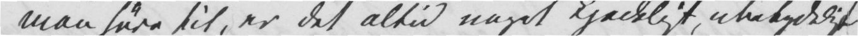man høre til, er det altid noget kjedeligt, ubetydeligt
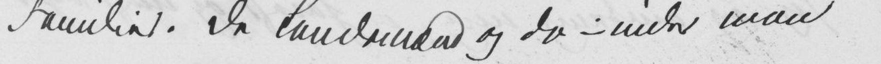Familier. De Landsmænd og do –inder man
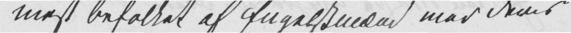mest befolket af Engelskmænd med deres
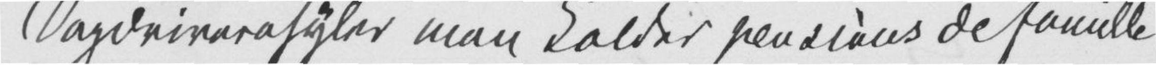Dagdriverasyler man kalder pensions de famille
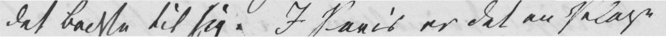det bedste til sig. I Paris er det en Plage
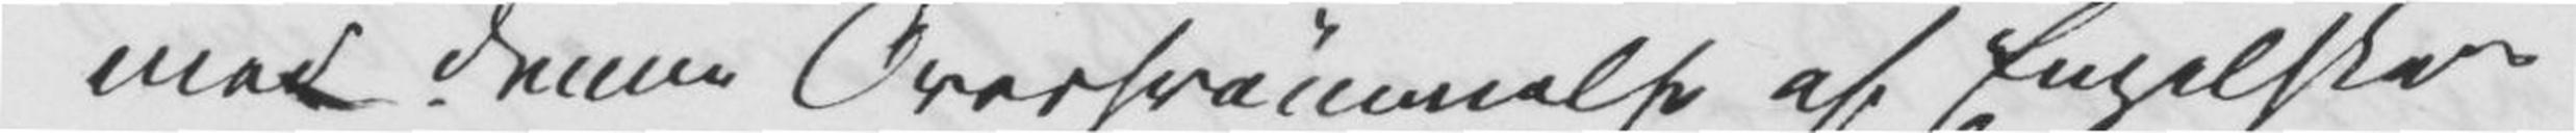med denne Oversvømmelse af Engelske
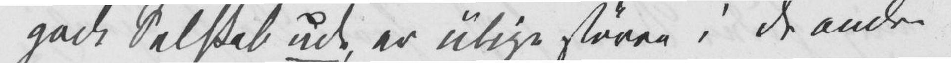godt Selskab ude, er ulige større i de andre
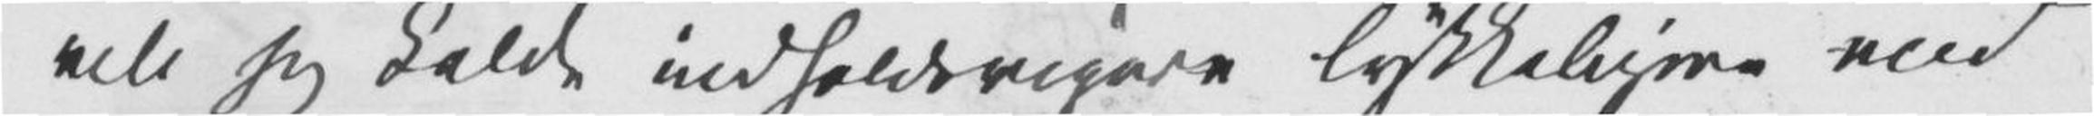vil jeg kalde indholdsrigere lykkeligere end
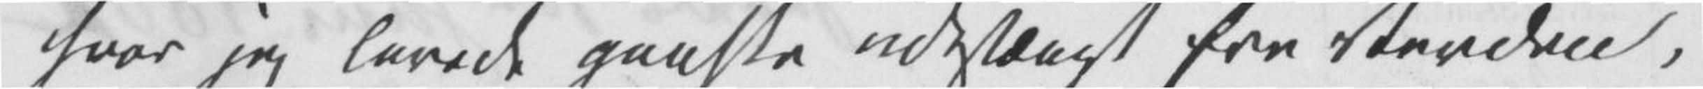hvor jeg levede ganske udestængt fra Verden,
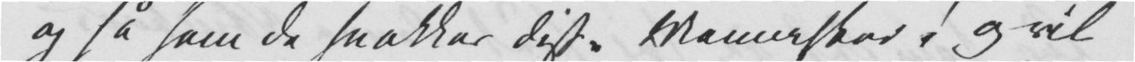og så som de snakker disse Mennesker! og vil
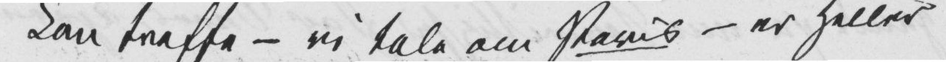kan treffe – vi tale om Paris – er heller
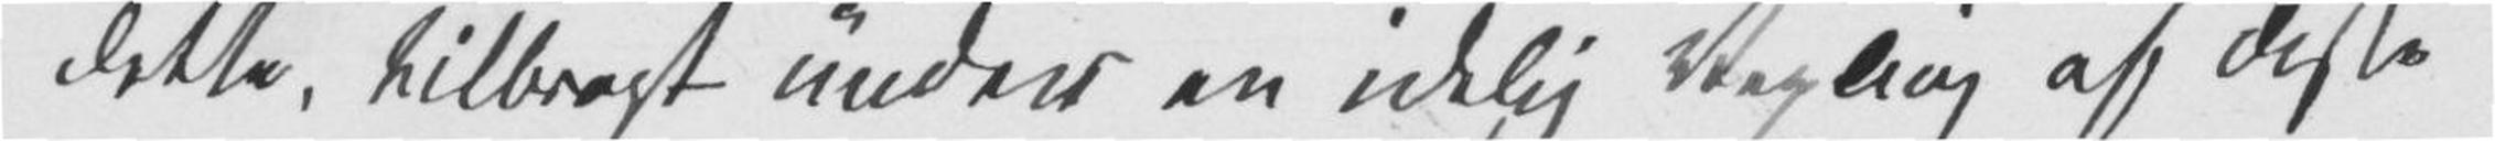dette, tilbragt under en idelig Vexling af disse
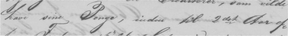have sine Penge, inden til 2det det Aar ef-
